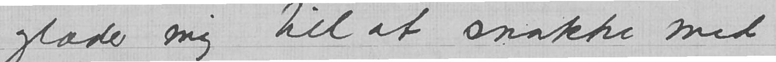Jeg glæder mig til at snakke med dig
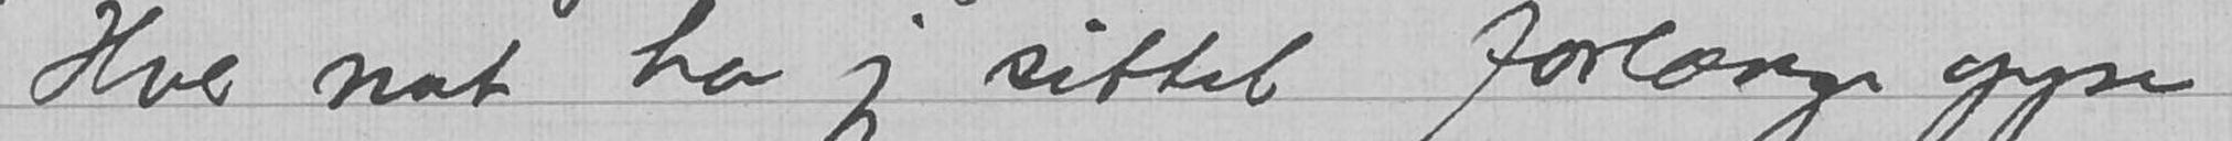Hver nat har jeg sittet forlænge oppe
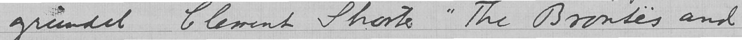grundet Clement Shorter «The Brontës and
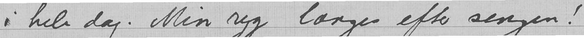i hele dag. Min ryg længes efter sengen!
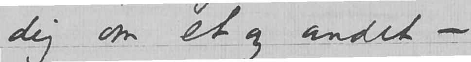om et og andet –.
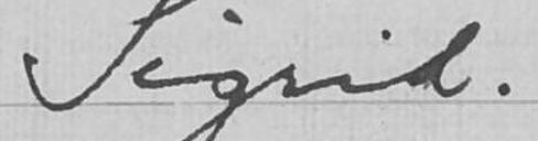Sigrid
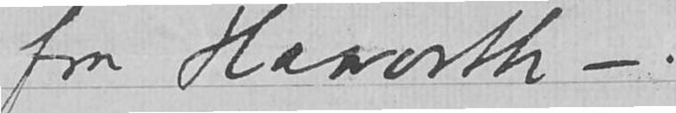fra Haworth –.
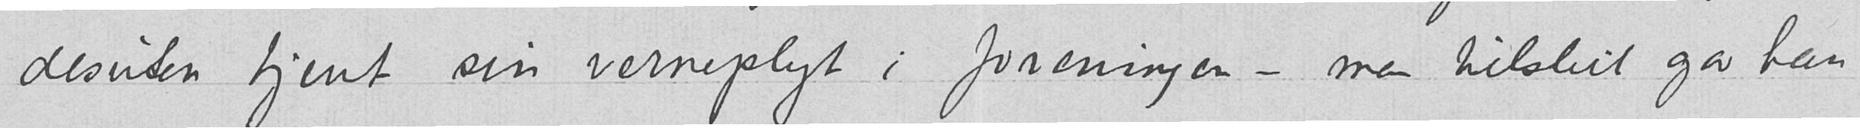desuten tjent sin vernepligt i foreningen – men tilslut gav han
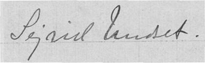Sigrid Undset.
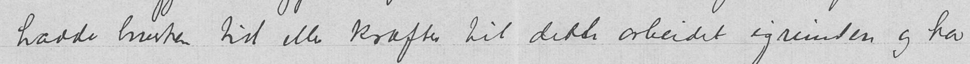hadde hverken tid eller kræfter til dette arbeidet igrunden og har
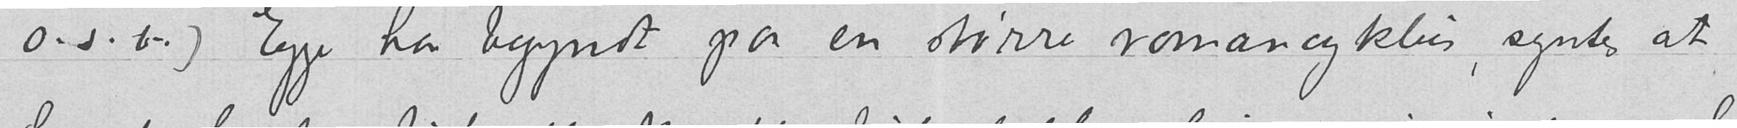o.s.v.) Egge har begyndt paa en større romancyklus, syntes at
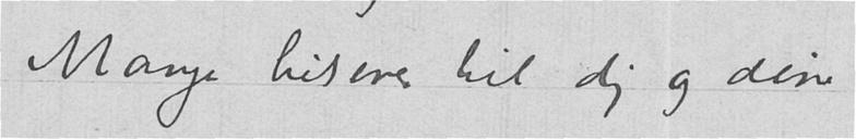Mange hilsener til dig og dine
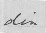din
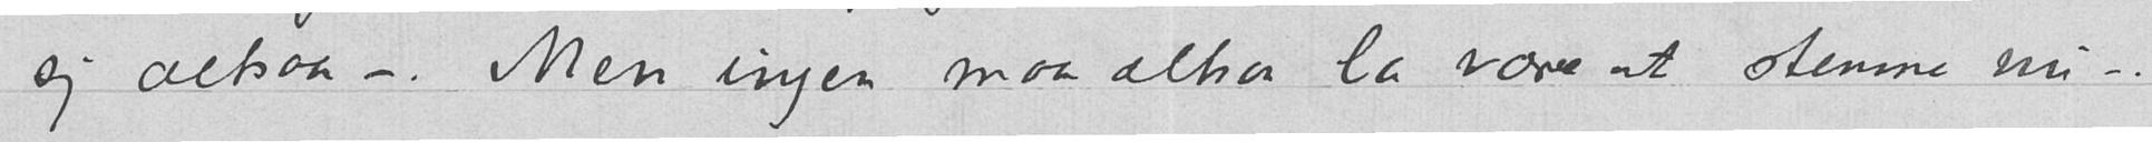sig altsaa –. Men ingen maa altsaa la være at stemme nu –.
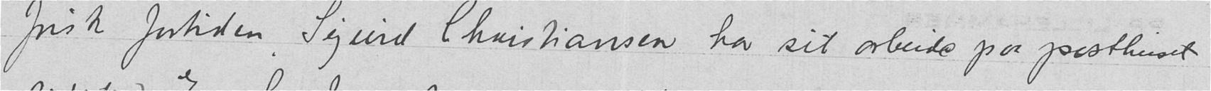frisk fortiden, Sigurd Christiansen har sit arbeide paa posthuset
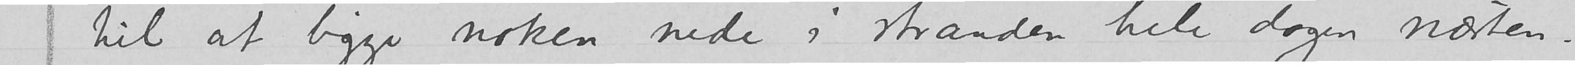til at ligge naken nede i stranden hele dagen næsten.
